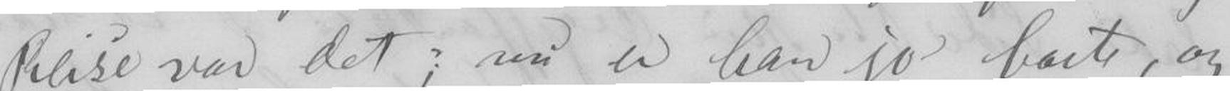Reise var det; nu er han jo borte, og
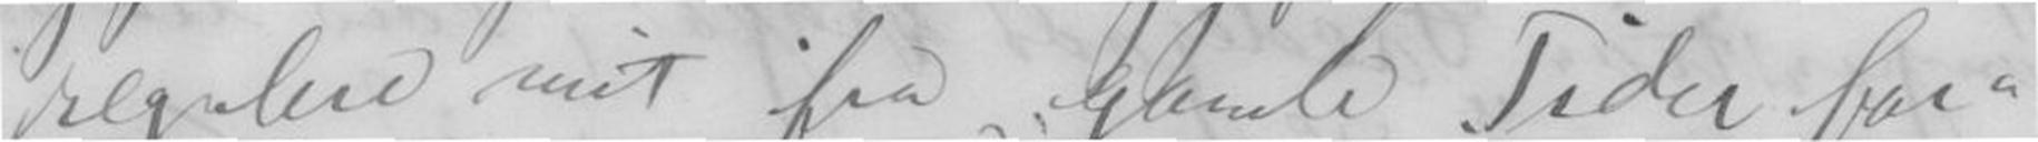regulere mit fra gamle Tider for-
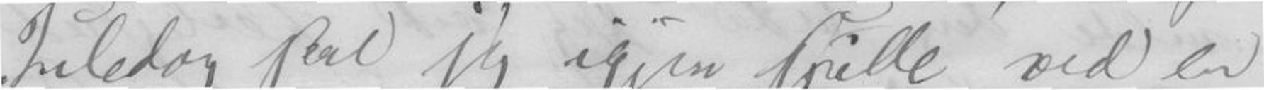Juledag skal jeg igjen spille ved en
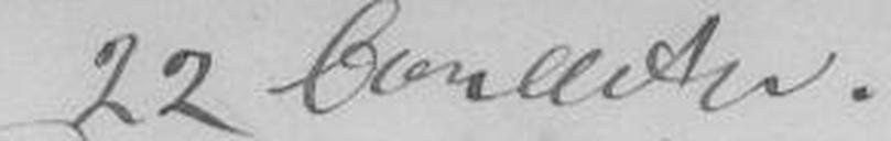22 Concerter
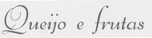Queijo e frutas
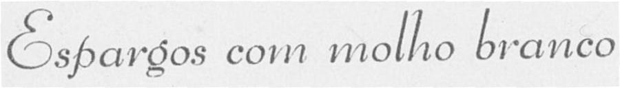Espargos com molho branco
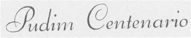Pudim Centenario
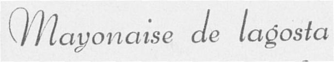Mayonaise de lagosta
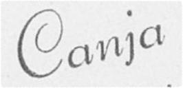Canja
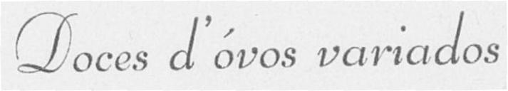Doces d'ovos variados
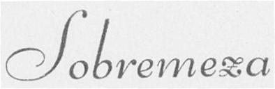Sobremeza
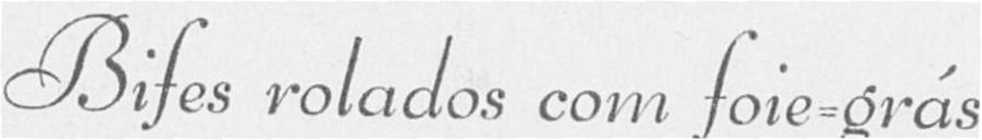Bifes rolados com foie-gras
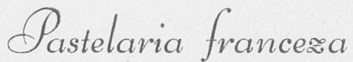Pastelaria franceza
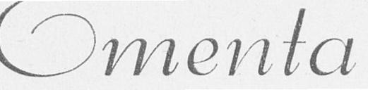Ementa
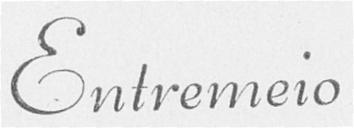Entremeio
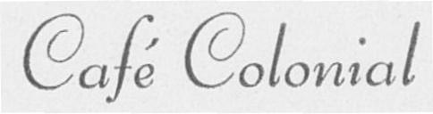Case Colomal
